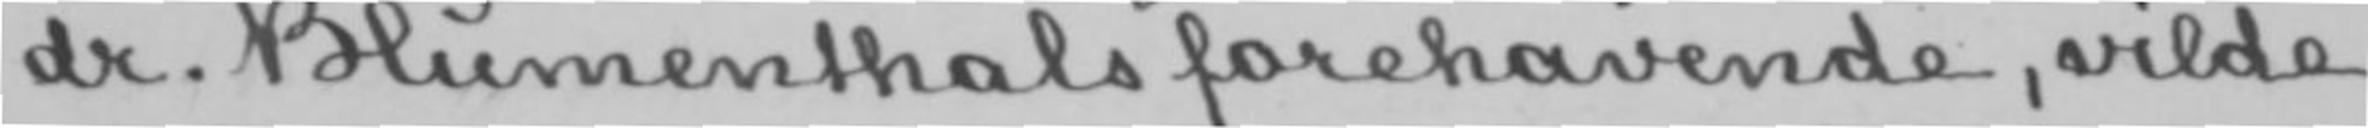dr. Blumenthals forehavende, vilde
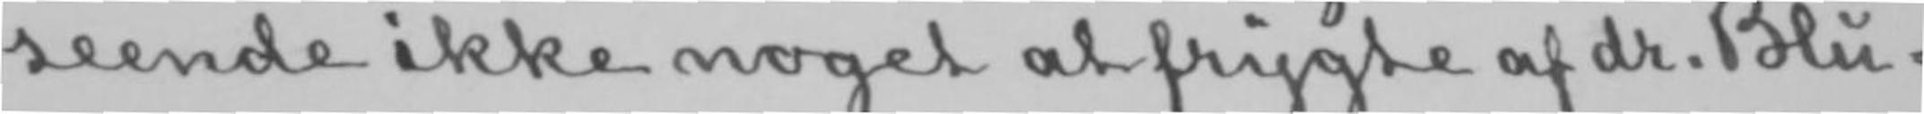seende ikke noget at frygte af dr. Blu-
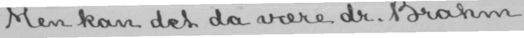Men kan det da være dr. Brahm
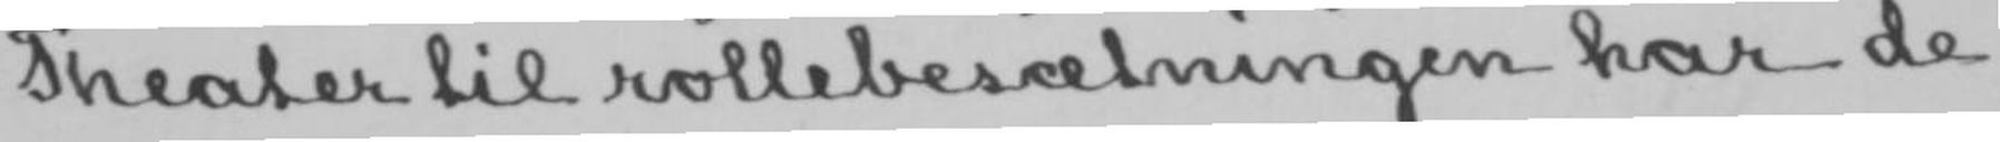Theater til rollebesætningen har de
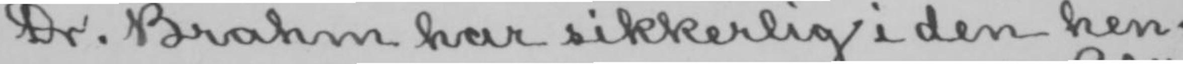Dr. Brahm har sikkerlig i den hen-
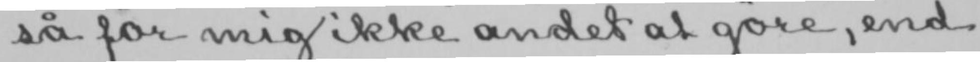så for mig ikke andet at gøre, end
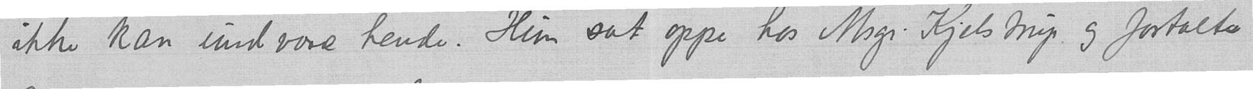ikke kan undvære hende. Hun sat oppe hos Msgr. Kjelstrup og fortalte
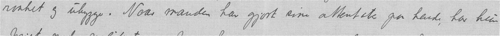raahet og uhygge. Naar manden har gjort sine attentater paa hende, har hun
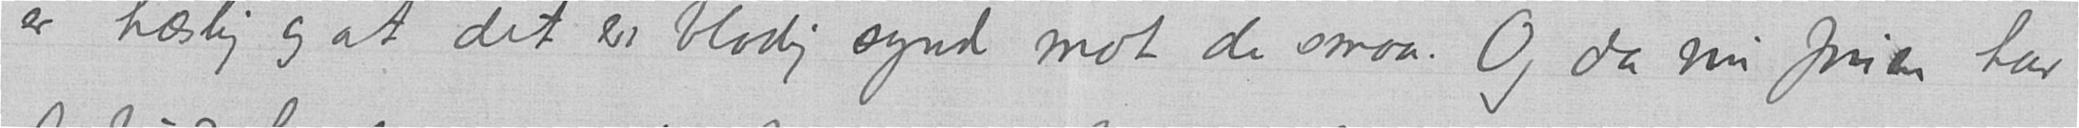er hæslig og at det er blodig synd mot de smaa. Og da nu fruen har
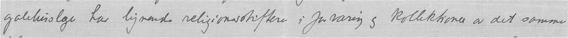galehuslæge har lignende religionssstiftere i forvaring og kollektioner av det samme
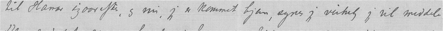til Hamar igaareftes, og nu, jeg er kommet hjem, synes jeg virkelig jeg vil meddele
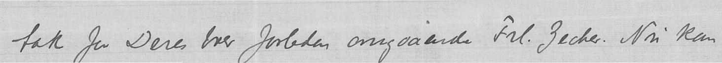tak for Deres brev forleden angående Frk. Zecher. Nu kan
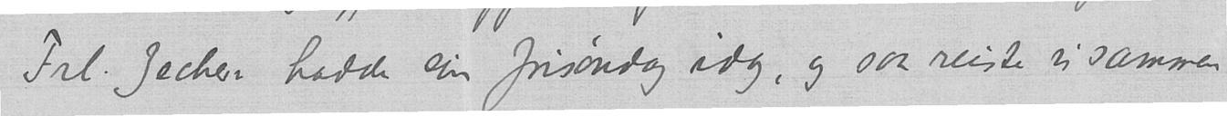Frk. Zecher hadde sin frisøndag idag, og saa reiste vi sammen
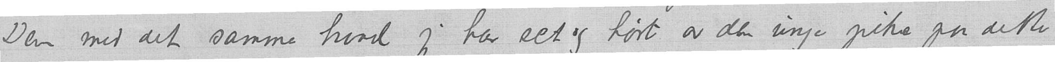Dem med det samme hvad jeg har set og hørt av den unge pike paa dette
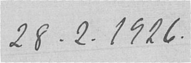28.2.1926.
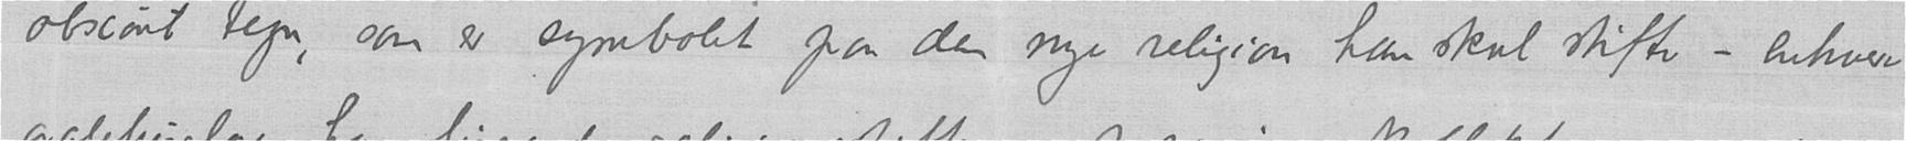obscønt tegn, som er symbolet paa den nye religion han skal stifte – enhver
